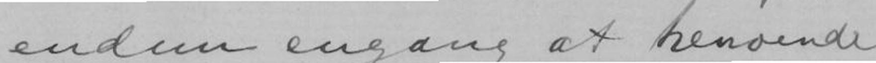endnu engang at henvende
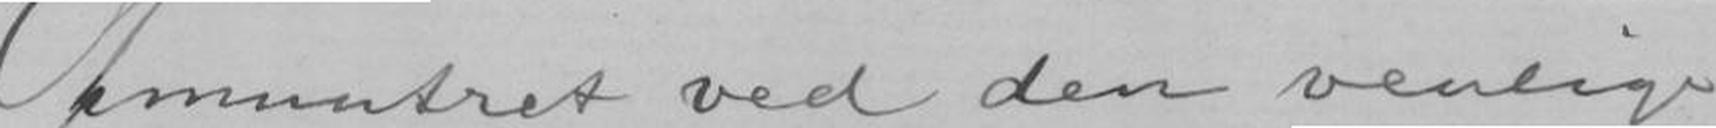Opmuntret ved den venlige
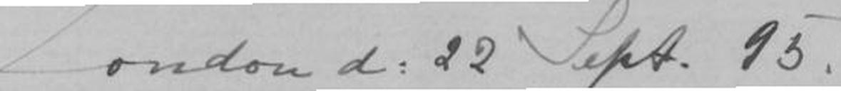London d: 22 Sept. 95.
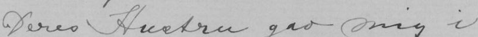Deres Hustru gav mig i
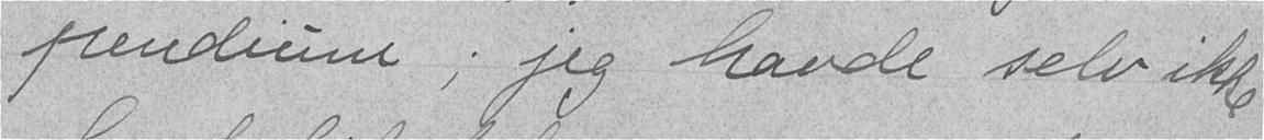pendium; jeg havde selv ikke
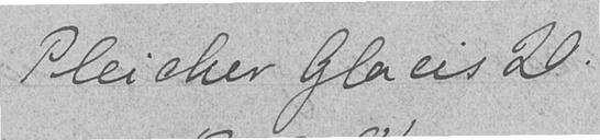Pleicher Glacis 20.
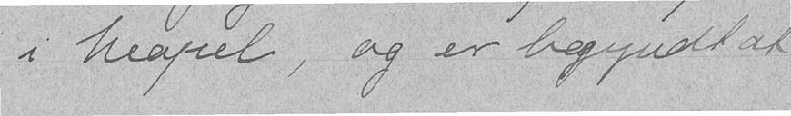i Neapel, og er begyndt at
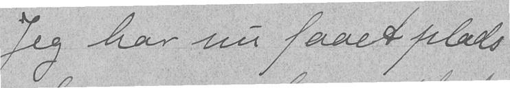Jeg har nu faaet plads
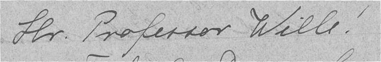Hr. Professor Wille!
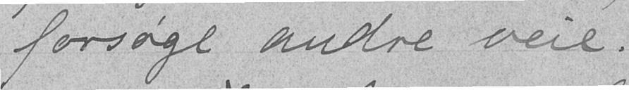forsøge andre veie.
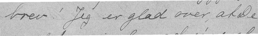brev! Jeg er glad over, at De
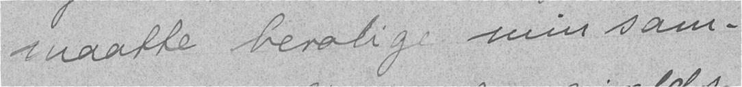maatte berolige min sam-
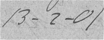13-2-01
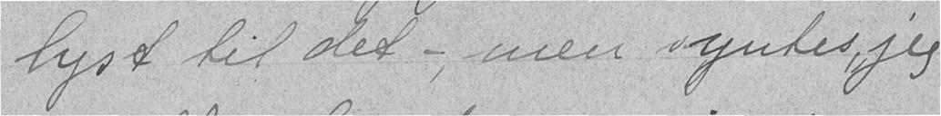lyst til det - men syntes, jeg
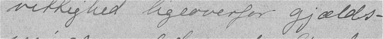vittighed ligeoverfor gjælds-
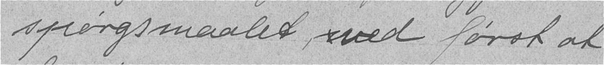spørgsmaalet, ved først at
